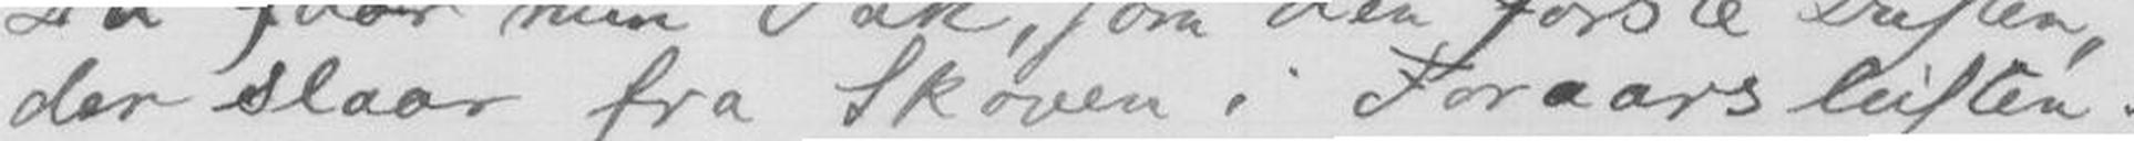der slaar fra Skoven i Foraarsluften.
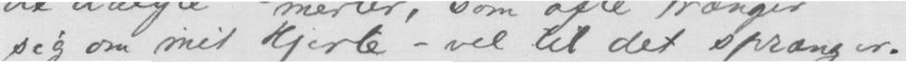sig om mit Hjerte - vel til det sprenger.
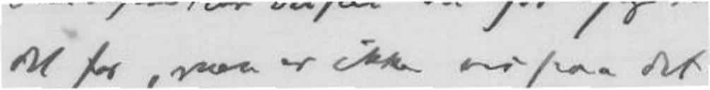det før, men er ikke ens paa det.
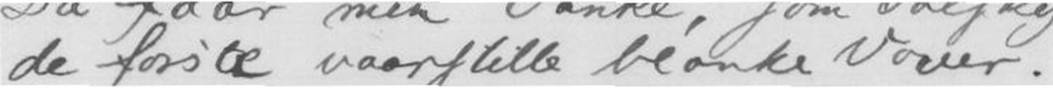de første vaarstille blanke Vover.
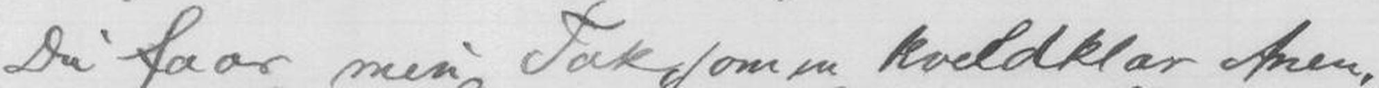Du faar min Tak, som en kveldklar Amen,
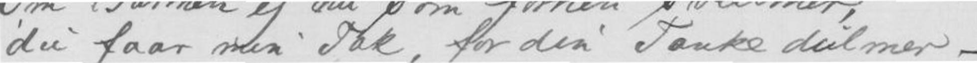du faar min Tak, for den Tanke dulmer -
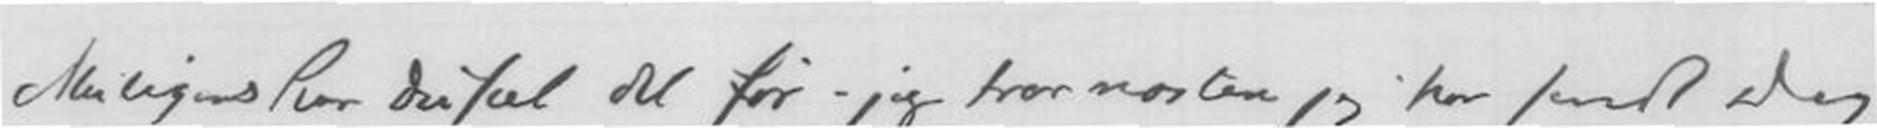Muligens har du faat det før - jeg tror næsten jeg har sendt dig
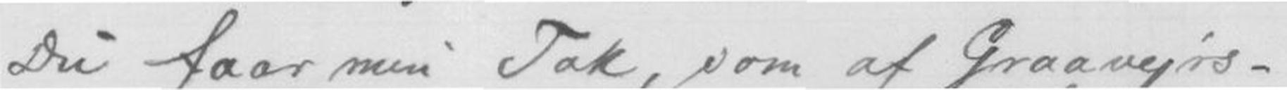Du faar min Tak, som af Graavejrsdunkling,
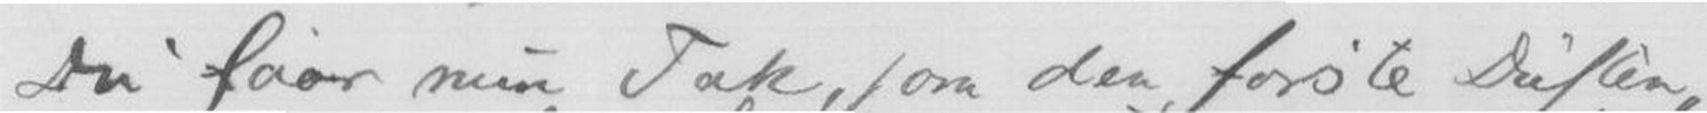Du faaar min Tak, som den første Duften,
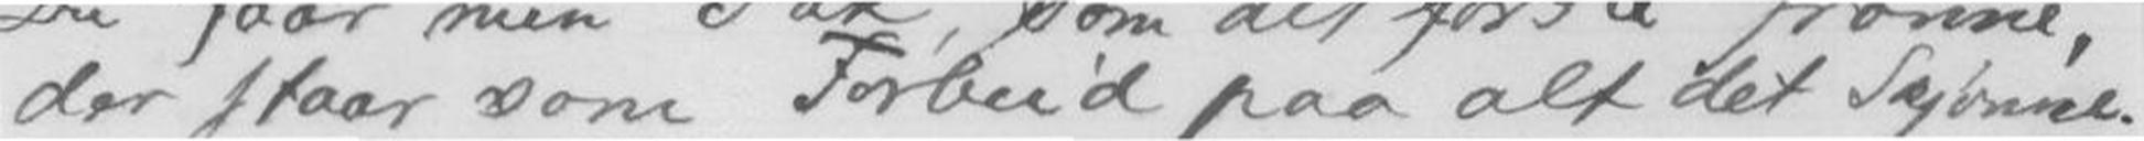der staar som Forbud paa alt det Skjønne.
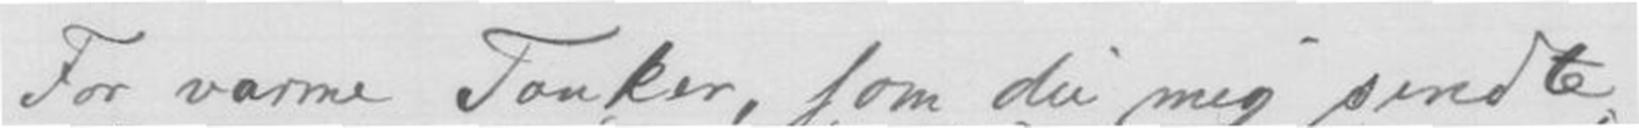For varme Tanker, som du mig sendte
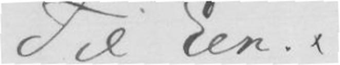Til Een.
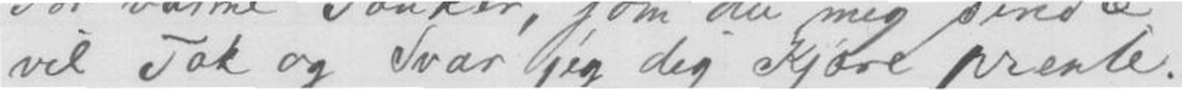vil Tak og Svar jeg dig Kjære prente.
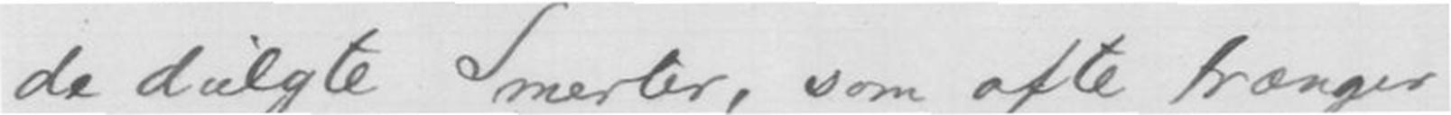de dulgte Smerter, som ofte trænger
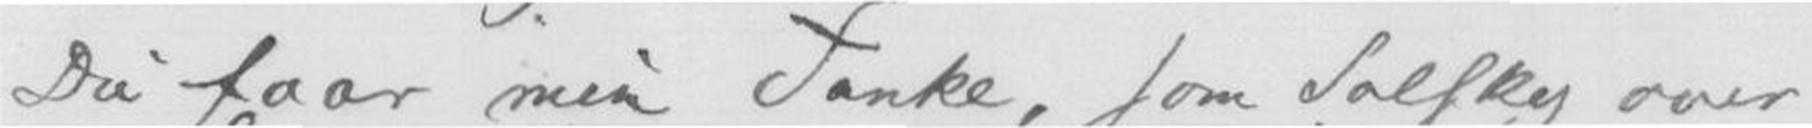Du faar min Tanke, som Solsky over
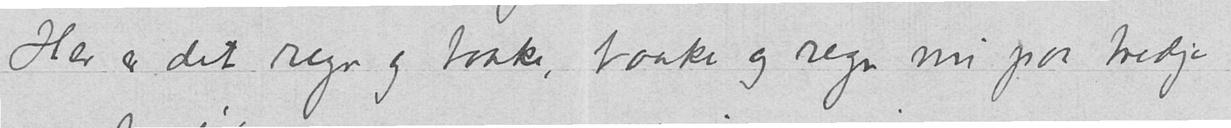Her er det regn og taake, taake og regn nu paa tredje
